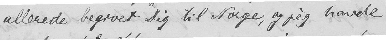allerede begivet Dig til Norge, og jeg havde
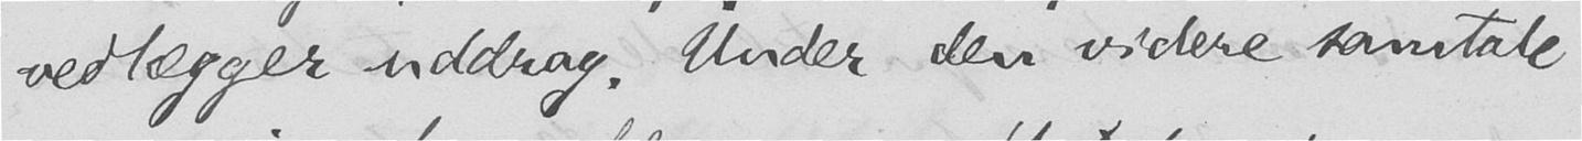vedlægger uddrag. Under den videre samtale
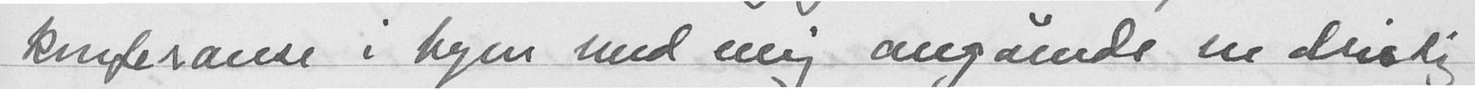konferanse i byen med mig angående en dristig
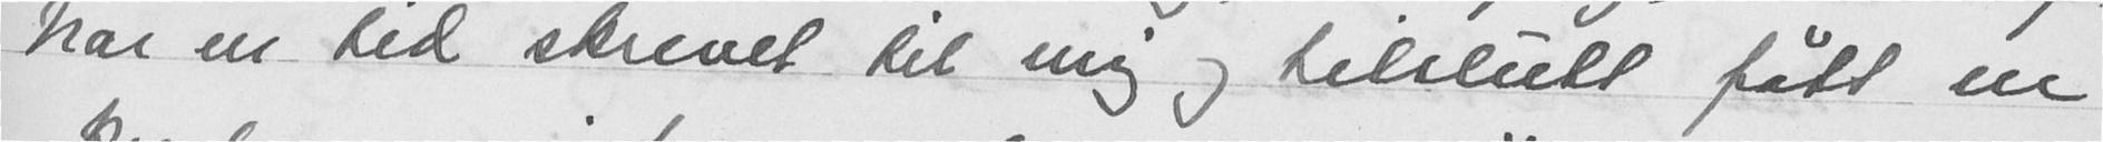har en tid skrevet til mig og tilslutt fått en
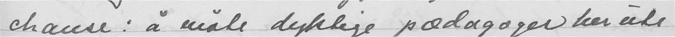chanse: å møte dyktige pædagager herute
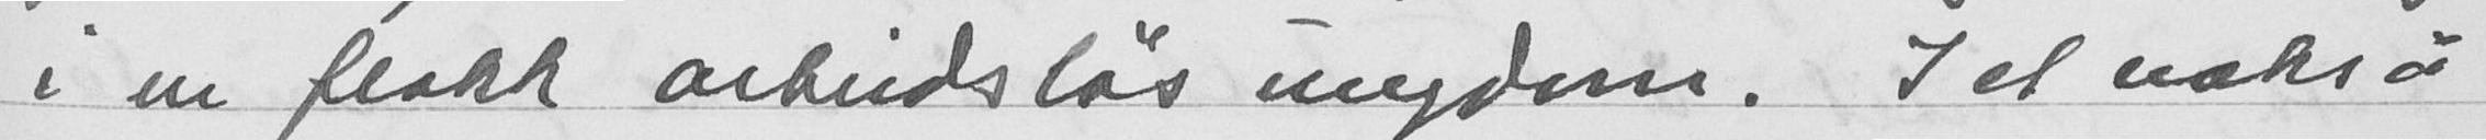i en flokk arbeidsløs ungdom. I et nokså
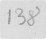138
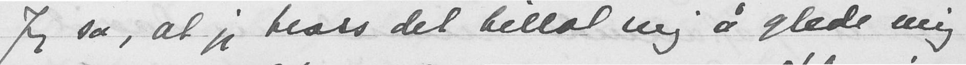Jeg sa, at jeg tross det tillot mig å glede mig
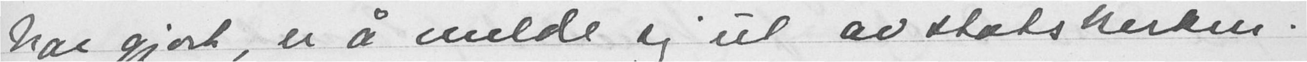har gjort, er å melde sig ut av statskirken.
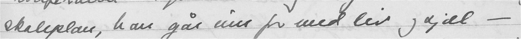skoleplan, han går inn for med liv og sjæl -
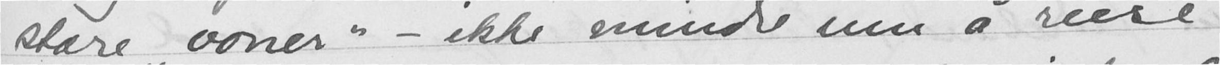store "voner" - ikke mindre enn å reise
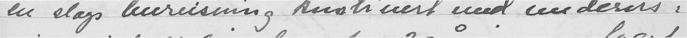en slags bureisning kurtisert med undervis-
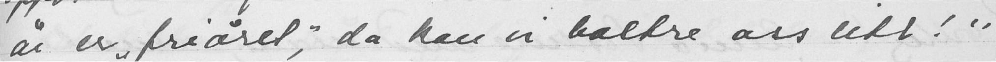år er "friåret", da kan vi boltre oss litt!"
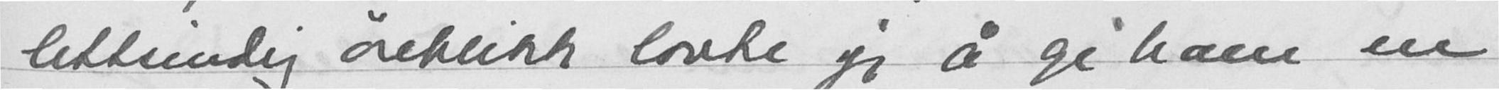lettsindig øieblikk lovte jeg å gi ham en
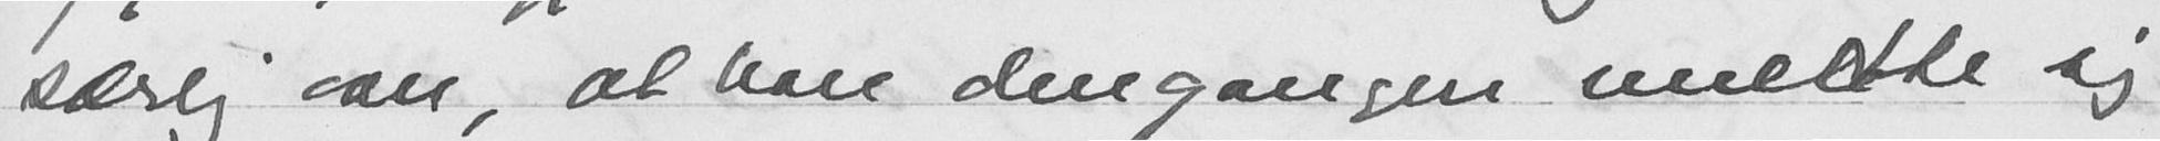særlig over, at han dengangen meldte sig
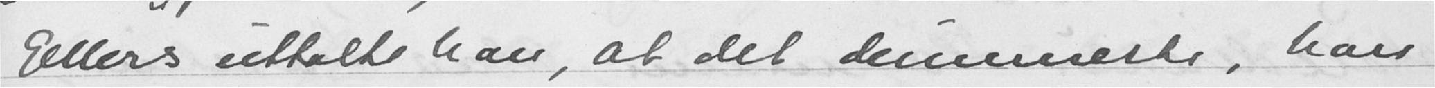Ellers uttalte han, at det dummeste, han
